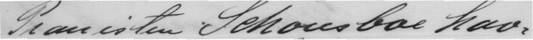Pianisten Schousboe hav-
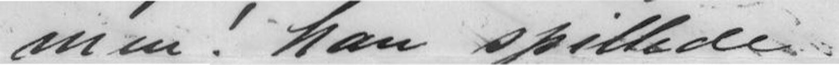men! han spillede
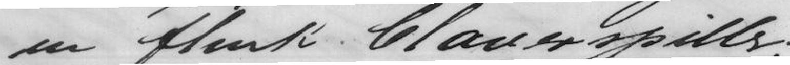en flink Claverspiller
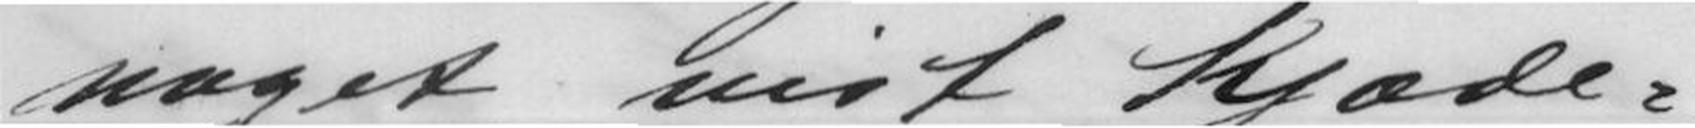noget vist kjæde-
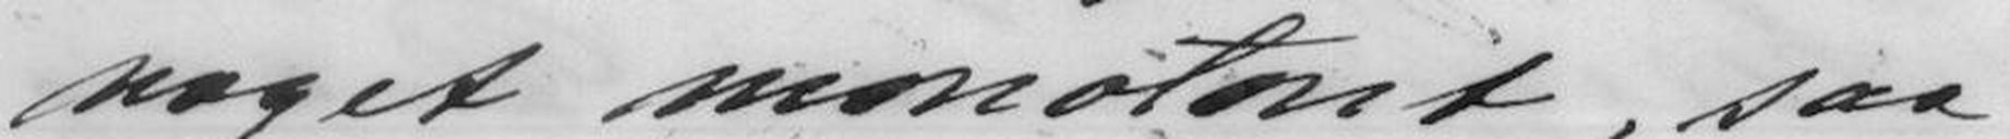noget monotont, saa
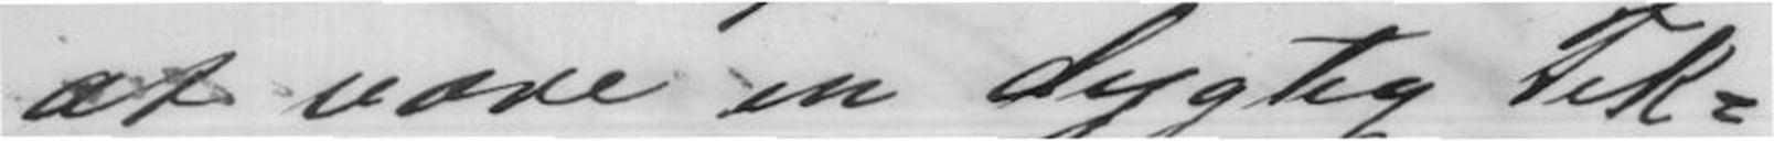at være en dygtig Tek-
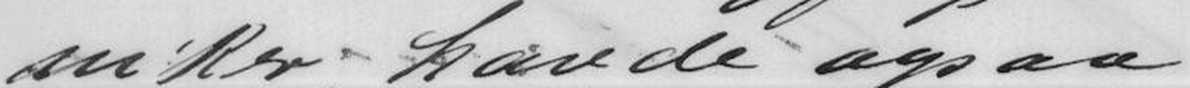niker; havde ogsaa
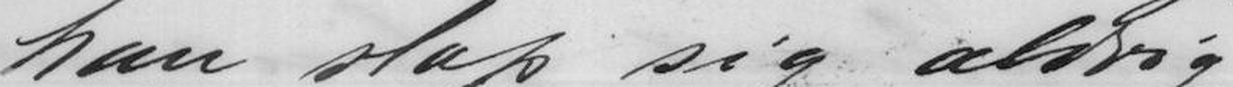han slap sig aldrig
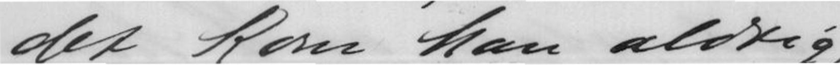det kom han aldrig
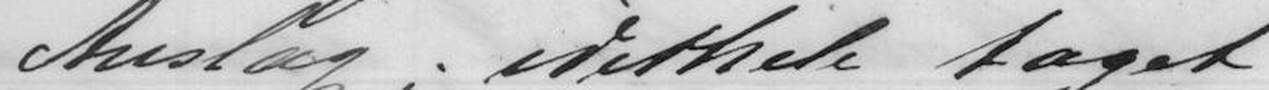Anslag; idethele taget
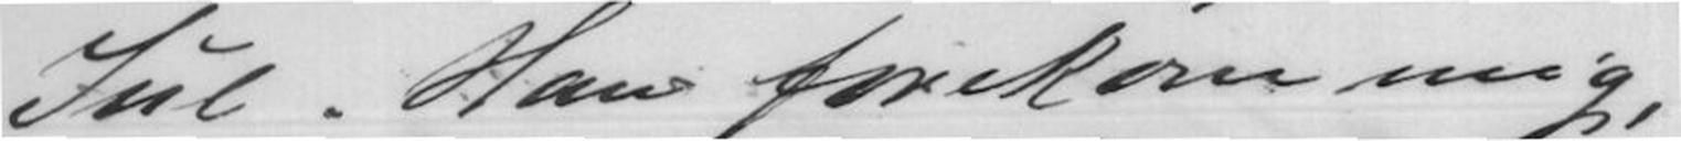Jul. Han forekom mig,
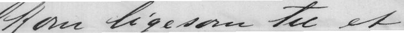kom ligesom til et
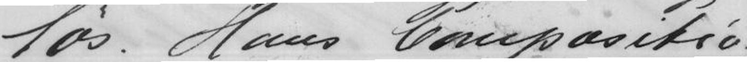løs. Hans Compositio
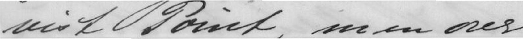vist Point, men over
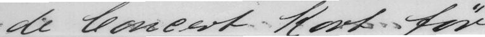de Concert kort før
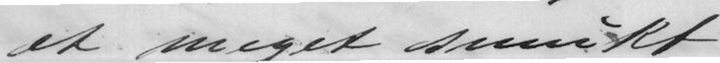et meget smukt
